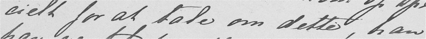cielt for at tale om dette, han
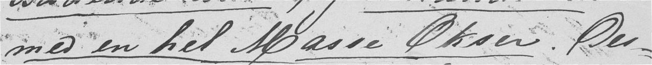med en hel Masse Okser. Des-
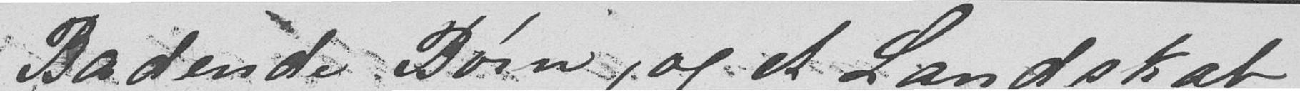Badende Børn, og et Landskab
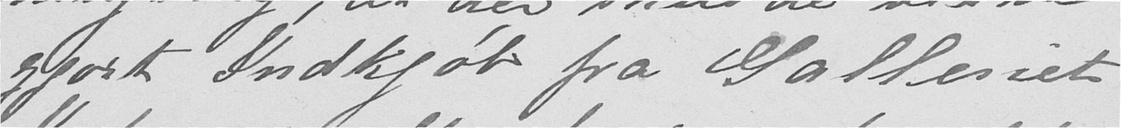gjort Indkjøb fra Galleriet.
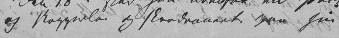og skoggerloe og skvadronerte paa sin
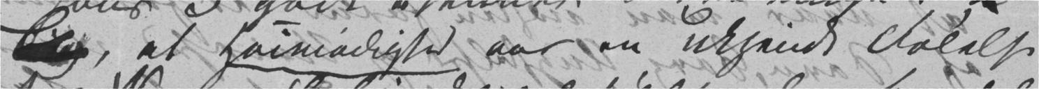Ting, at Høimodighed var en ukjendt Følelse
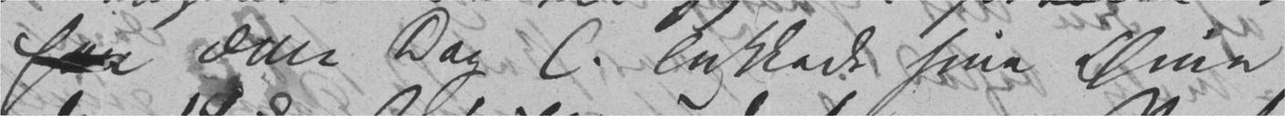han den Dag C. lukkede sine Øine
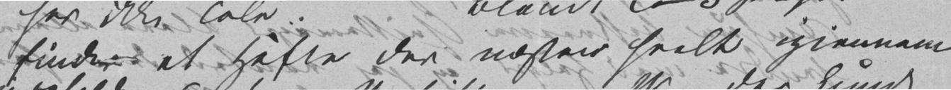findes et Hefte der næsten heelt igjennem
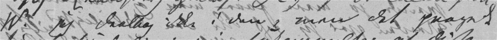W. jeg deeltog ikke i den, men det prægede
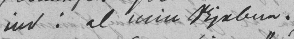ind i al min Skjebne.
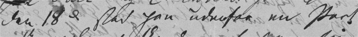den 18de stod han udenfor en Port
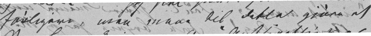førligere, men maae til dette gjøre et
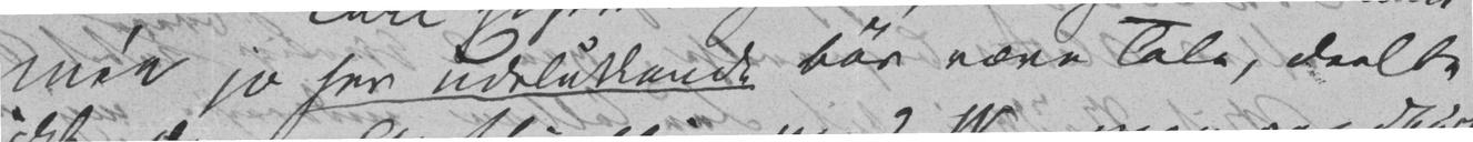mée jo her udelukkende bør være Tale, deelte
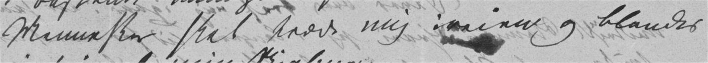Menneske skal træde mig iveien og blandes
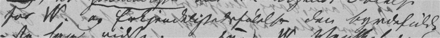for W. og Erkjendtlighedsfølelse den byrdefulde
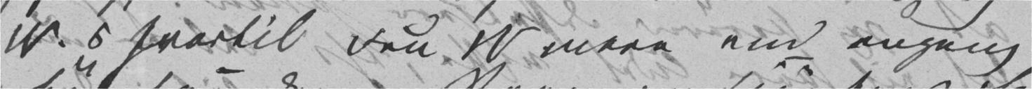W.s hvortil Fru W mer end engang
